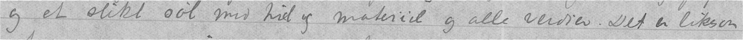og et slikt søl med til og materiel og alle verdier. Det er liksom
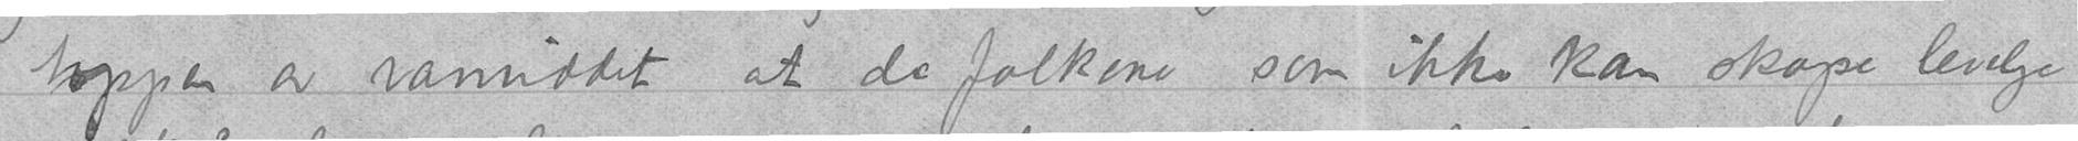toppen av vanviddet at de folkene som ikke kan skape levelige
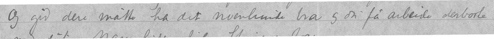Og gid dere måtte ha det noenlunde bra og du få arbeide derborte
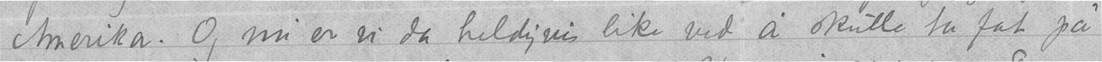Amerika. Og nu er vi da heldigvis like ved å skulle ta fat på
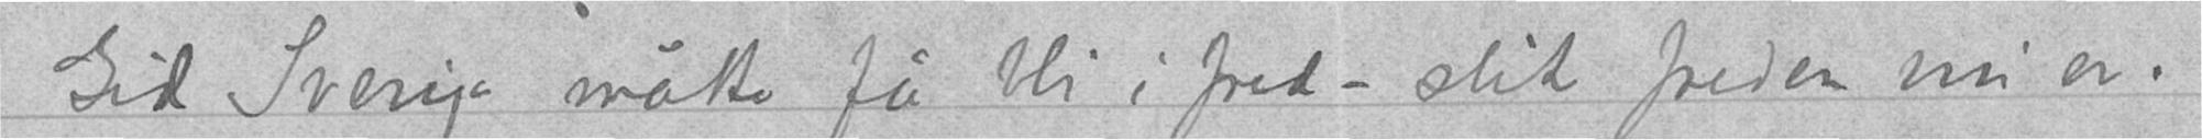Gid Sverige måtte få bli i fred – slik freden nu er.
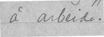å arbeide.
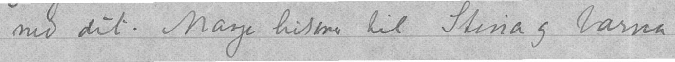med dit. Mange hilsener til Stina og barna
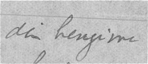din hengivne
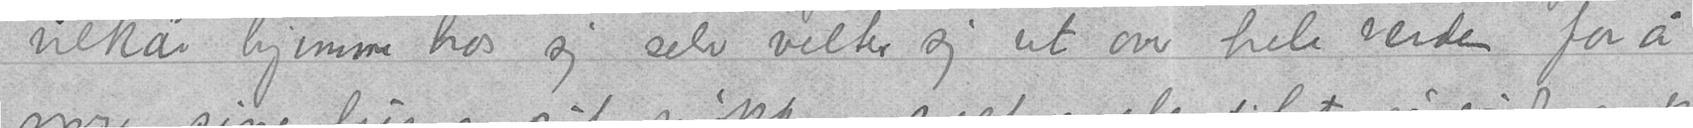vilkår hjemme hos sig selv velter sig ut over hele verden for å
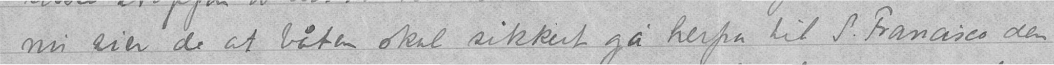nu sier de at båten skal sikkert gå herfa til S. Francisco den
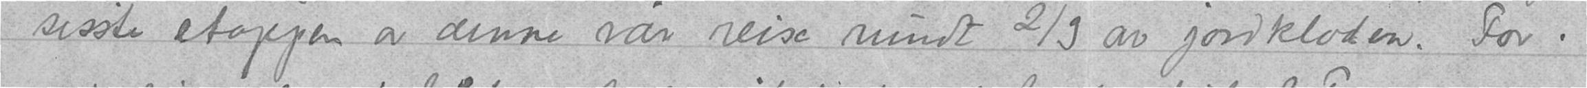sisste etappen av denne vår reise rundt 2/3 av jordkloden. For
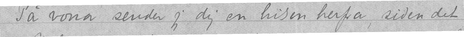På vona sender jeg dig en hilsen herfra, siden det
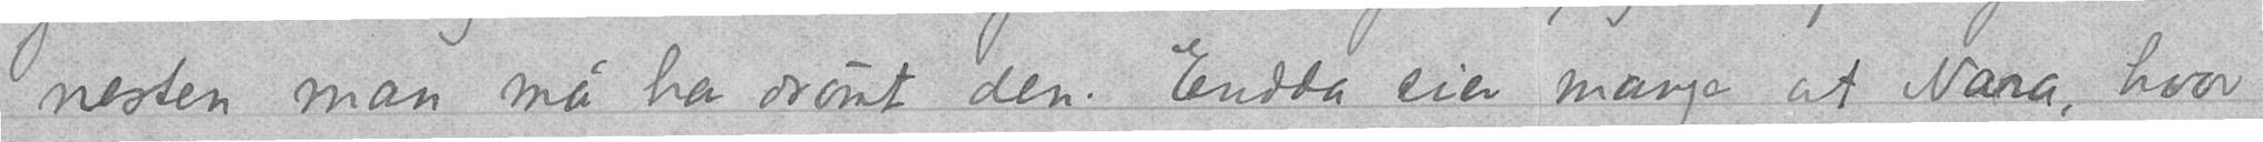nesten man må ha drømt den. Endda sier mange at Nara, hvor
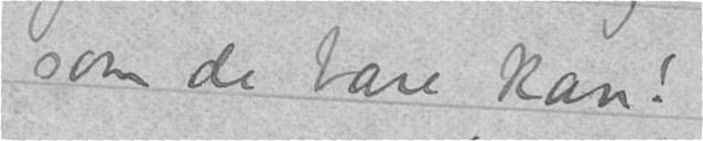som de bare kan!
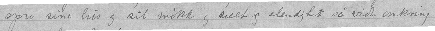spre sine lus og sit møkk og sult og elendighet så vidt omkring
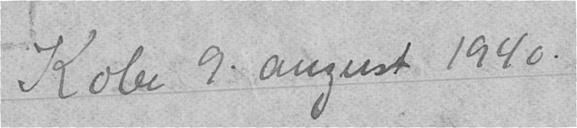Kobe 9. august 1940.
